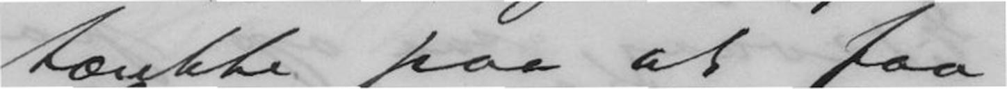tænkte paa at faa
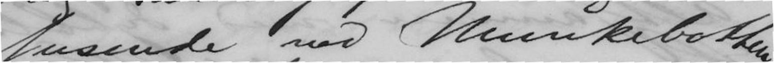susende ned Munkebotten,
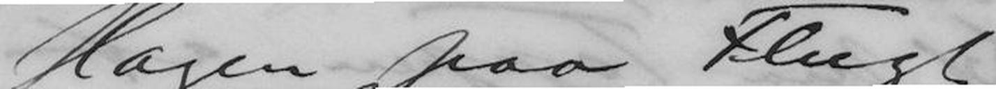slagen paa Flugt!
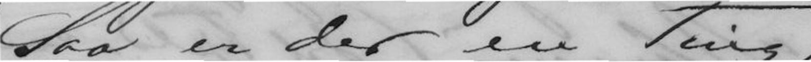Saa er der en Ting,
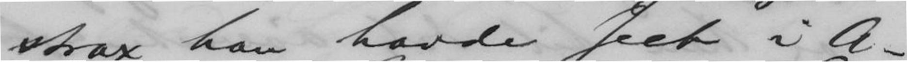strax han havde seet i A-
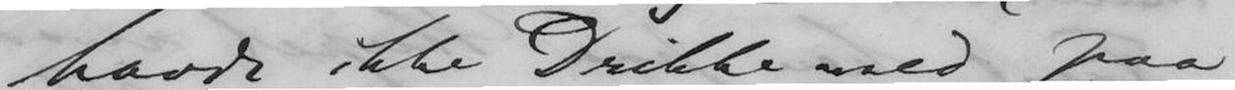havde ikke Drikke med paa
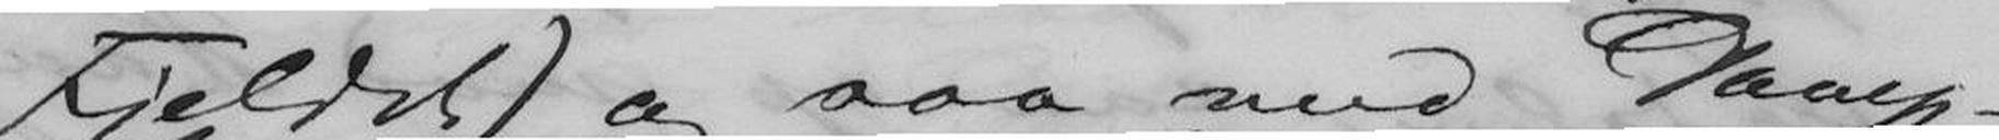Fjeldet) og saa med Damp-
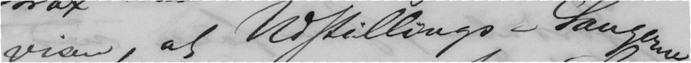visen, at Udstillings-Sangerne
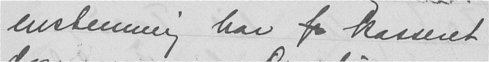enstemmig har fo kasseret
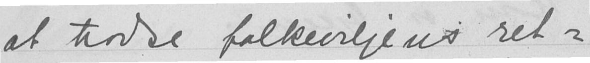at trodse folkeviljens ret-
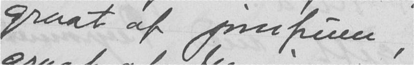graat af jomfruen,
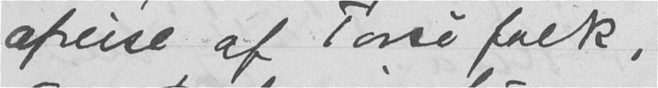afreise af Torsø folk,
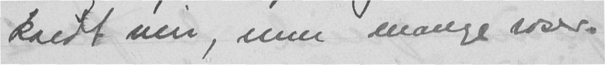koldt veir, men mange roser.
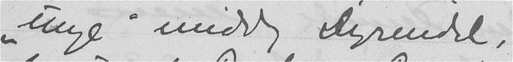"unge" middag Dyrendal,
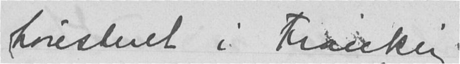høiesteret i Frankrig
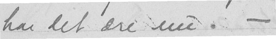har det ære nu. -
